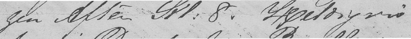gen Aften Kl. 8. Heldigvis
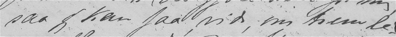saa jeg kan faa vide, om hun le-
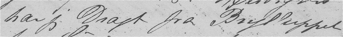har jeg Dragt fra Brylluppet
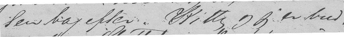len bagefter. Kitty og jeg er bud-
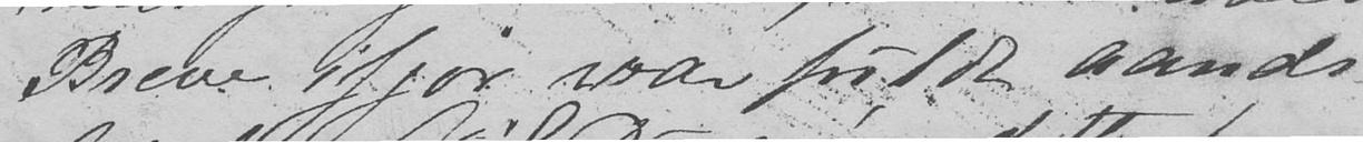Breve ifjor var fuldt aands-
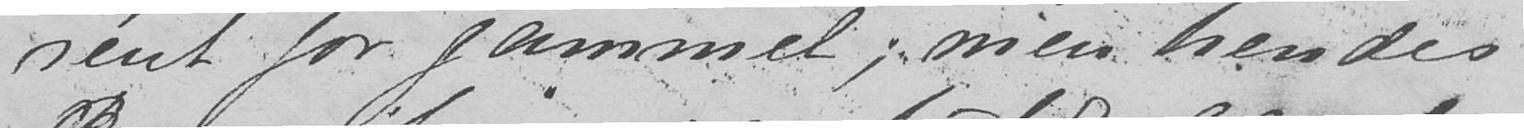rent for gammel; men hendes
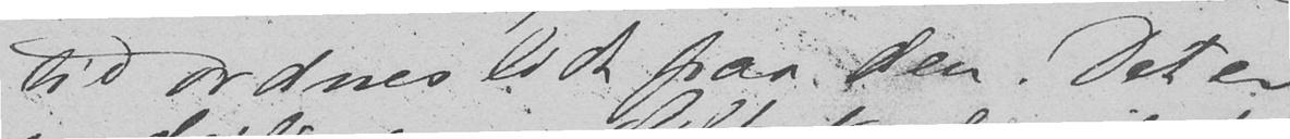tid ordnes lidt paa den. Det er
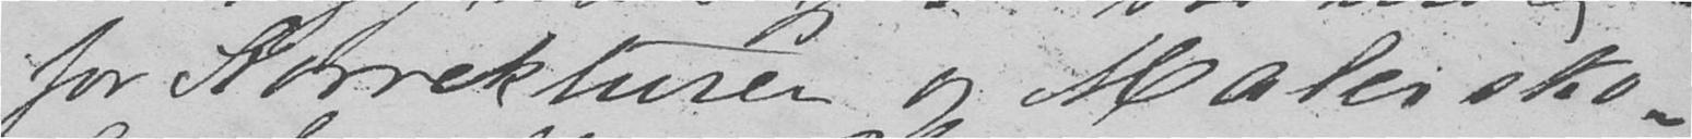for Korrekturen og Malersko-
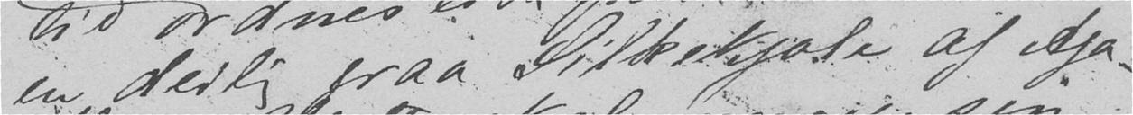en deilig graa Silkekjole af Aga-
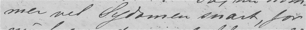mer vel Sydamen snart, for
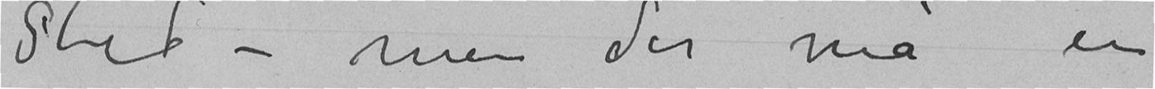Sted – men der må en
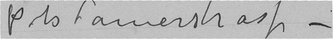Potsdamerstrasse –
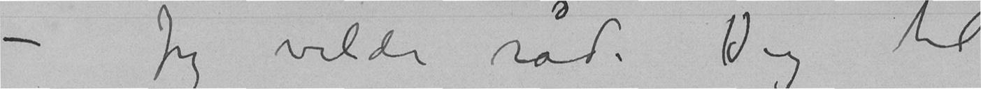– Jeg vilde råde Dig til
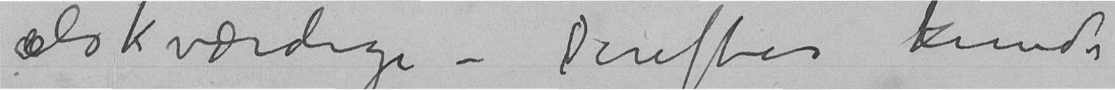elskværdige – Derefter kunde
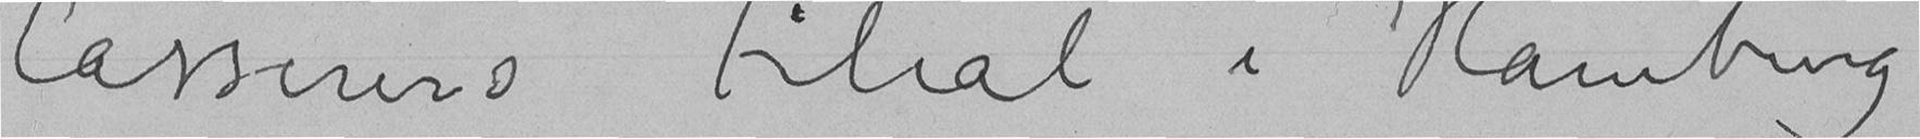Cassirers Filial i Hamburg –
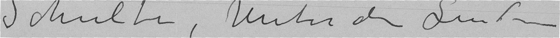Schulte, Unter den Linden
